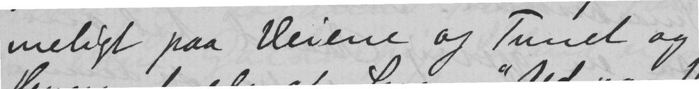meligt paa veiene og Tunet og
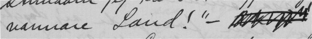varmare Land! -
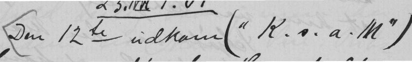Den 12te udkom ("K. s. a. M")
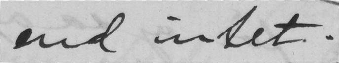end intet.
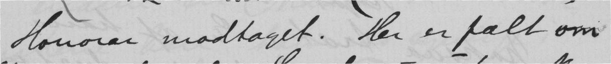Honorar modtaget. Her er fælt om
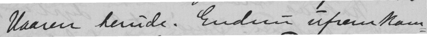Vaaren herude. Endnu ufremkom-
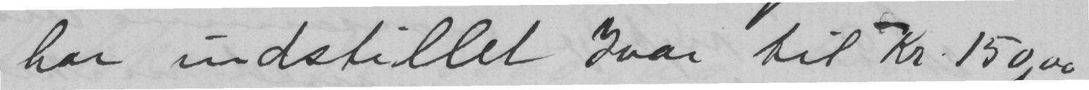har indstillet Ivar til Kr. 150,00
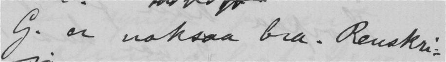G. er noksaa bra. Renskriv-
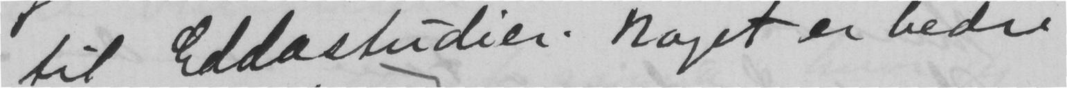til Eddastudier. noget er bedre
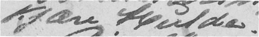Kjære Hulda.
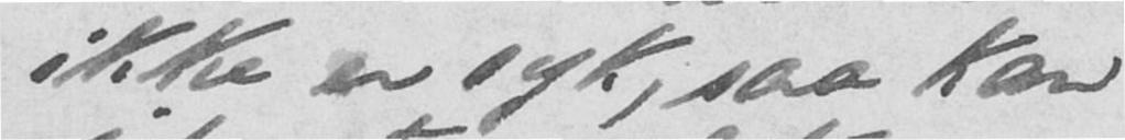ikke er syk, saa kan
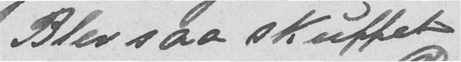Blev saa skuffet
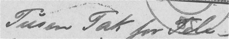Tusen Tak for Tele-
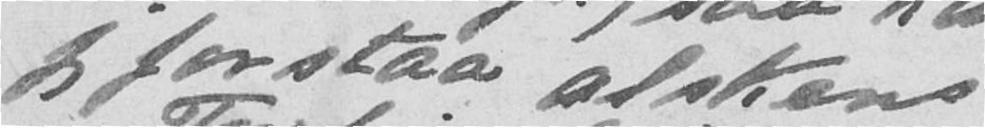jeg forstaa alskens
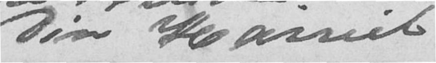Din Harriet
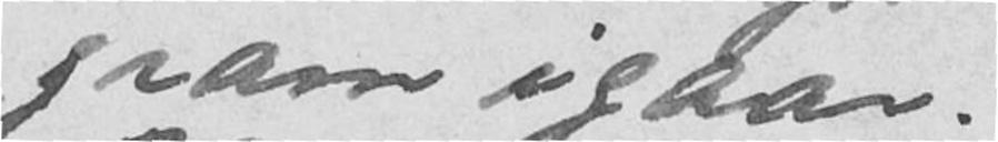gram igaar.
